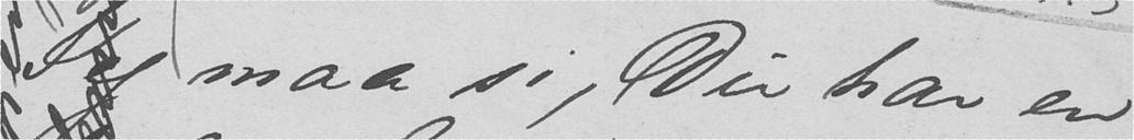Jeg maa si, Du har en
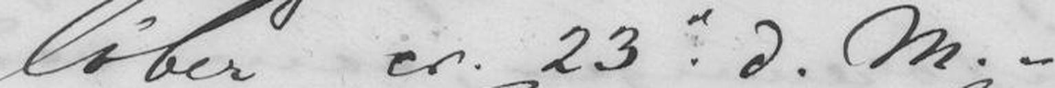løber c. 23de d. M. -
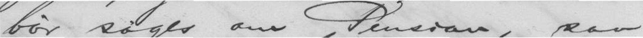bør søges om Pension, saa
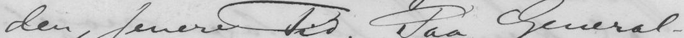den senere Tid. Paa General-
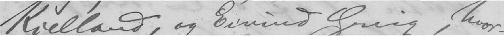Kielland, og Eivind Grieg, hvor-
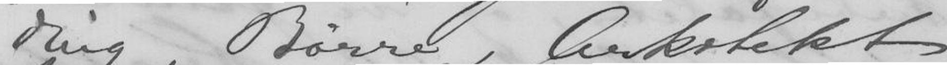ding, Børre, Arkitekt
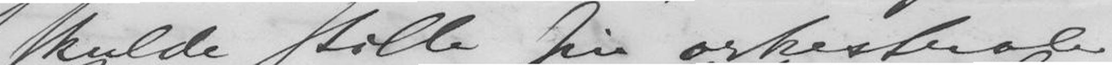skulde stille sin orkestrale
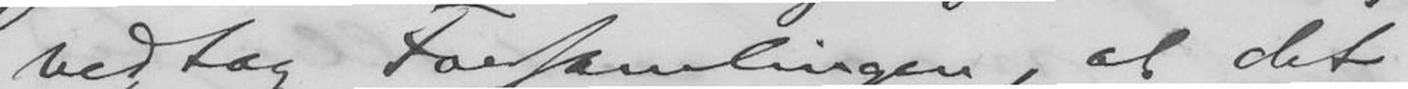vedtog Forsamlingen, at det
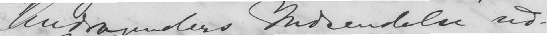Andragenders Indsendelse ud-
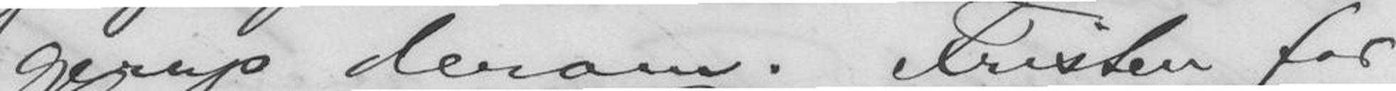gerup derom. Fristen for
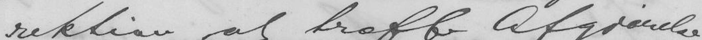rektion at træffe Afgjørelse
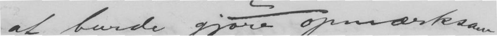at burde gjøre opmærksom
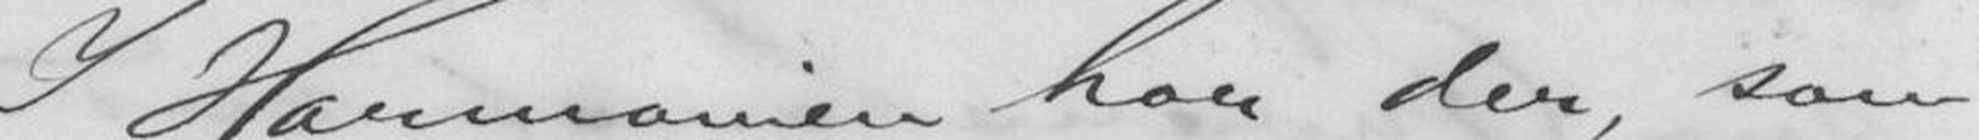I Harmonien har der, som
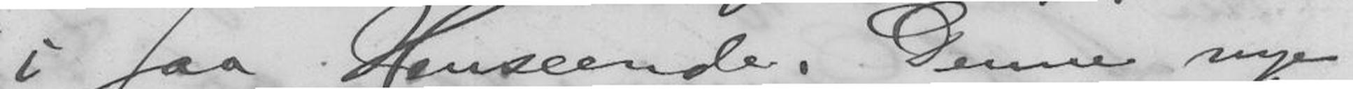i saa Henseende. Denne nye
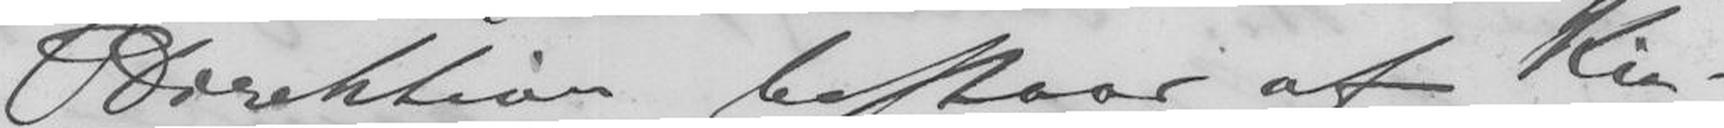Direktion bestaar af Kin-
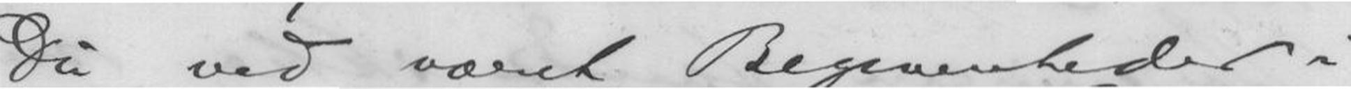Du ved været Begivenheder i
